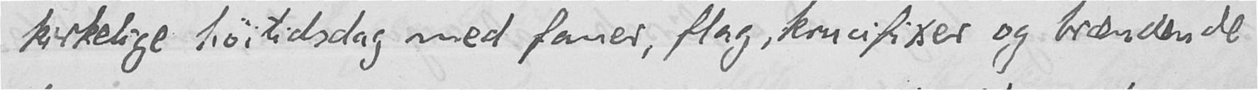kirkelige høitidsdag med faner, flag, krucifixer og brændende
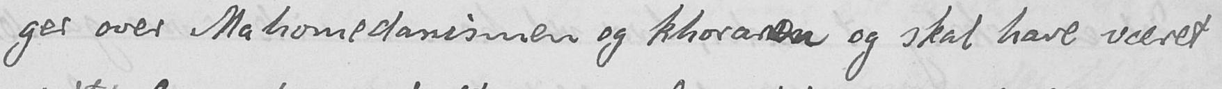ger over Mahomedanismen og khoranen og skal have været
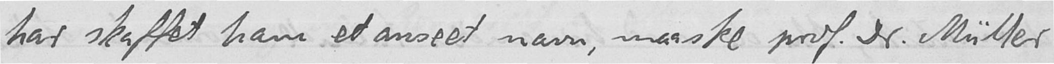har skaffet ham et anseet navn, maaske prof. Dr. Müller
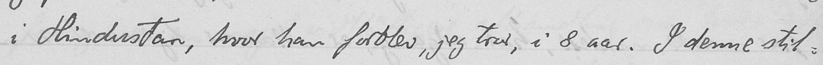i Hindustan, hvor han forblev, jeg tror, i 8 aar. I denne stil-
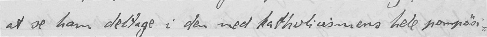at se ham deltage i den med katholicismens hele pompøsi-
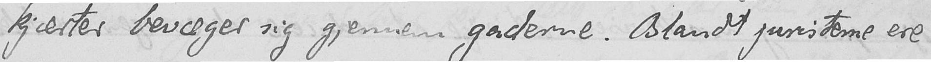kjærter bevæger sig gjennem gaderne. Blandt juristerne ere
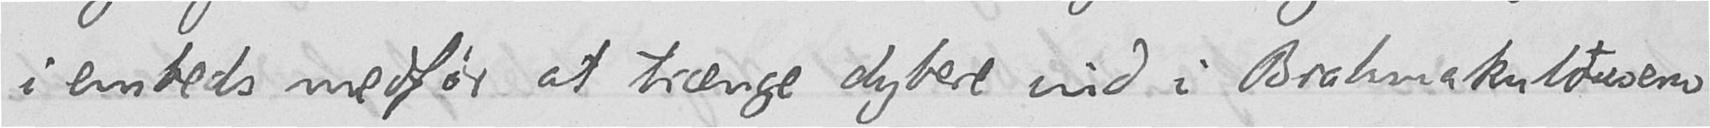i embeds medfør at trænge dybere ind i Brahmakultusens
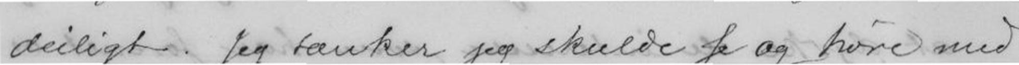deiligt. Jeg tænker jeg skulde se og høre med
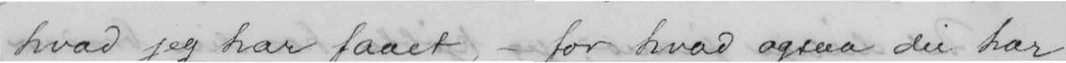hvad jeg har faaet, - for hvad ogsaa du har
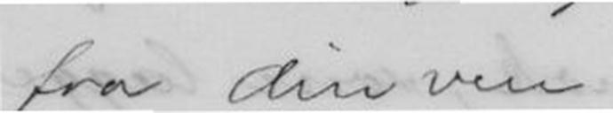fra din ven
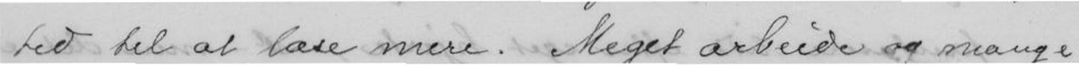tid til at læse mere. Meget arbeide og mange
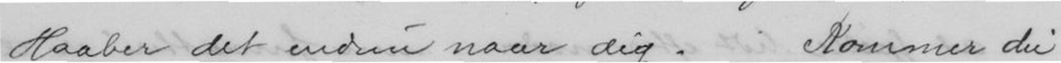Haaber det endnu naar dig. Kommer du
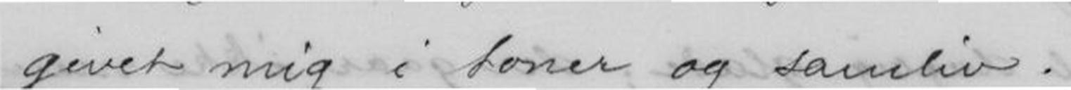givet mig i toner og samliv.
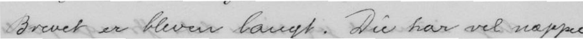Brevet er bleven langt. Du har vel næppe
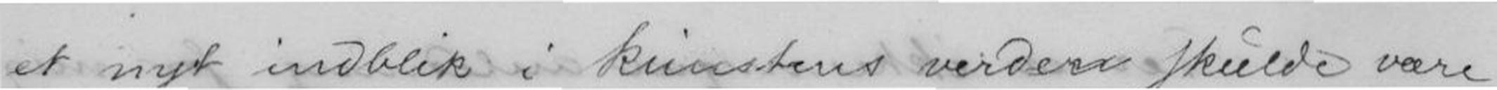et nyt indblik i kunstens verden skulde være
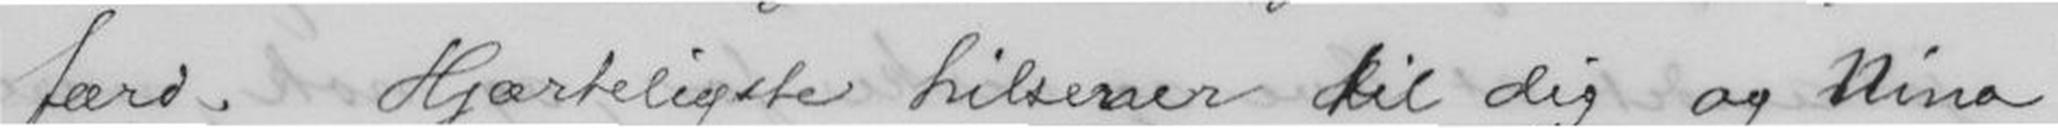færd. Hjærteligste hilsener til dig og Nina
